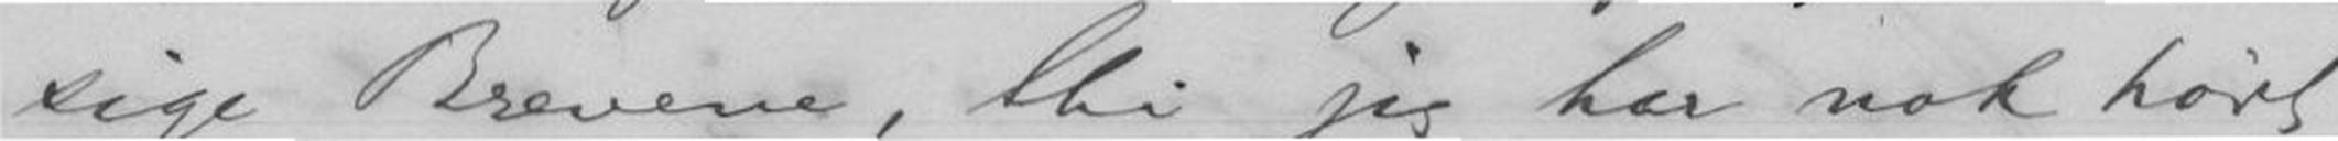sige Brevene, thi jeg har nok hørt
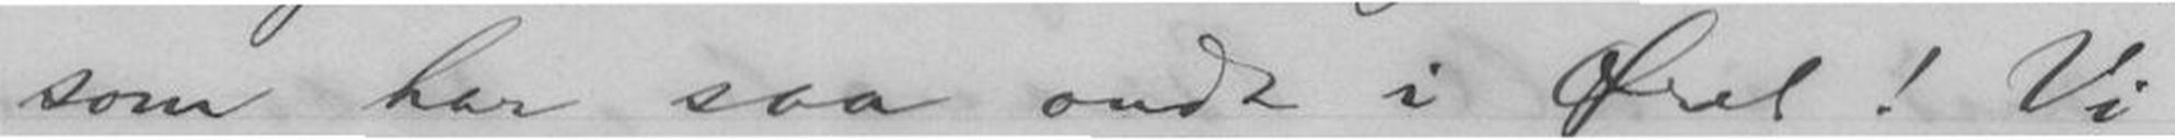som har saa ondt i Øiet! Vi
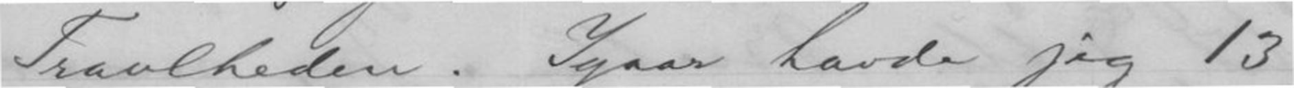Travlheden. Igaar havde jeg 13
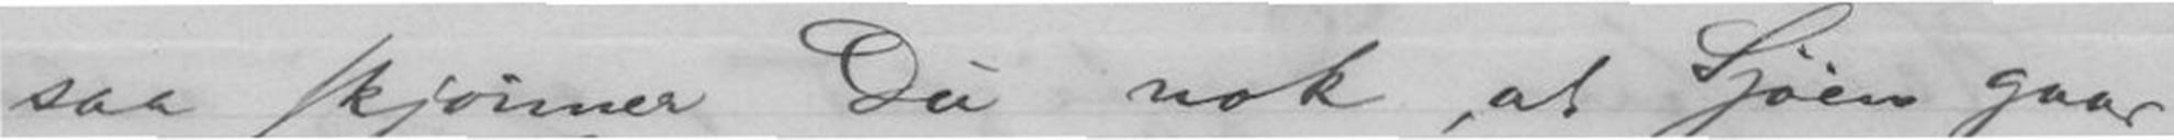saa skjønner Du nok, at Sjøen gaar
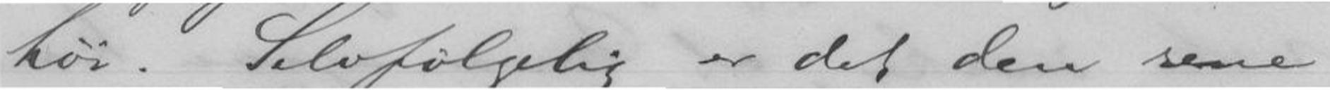høi. Selvfølgelig er det den rene
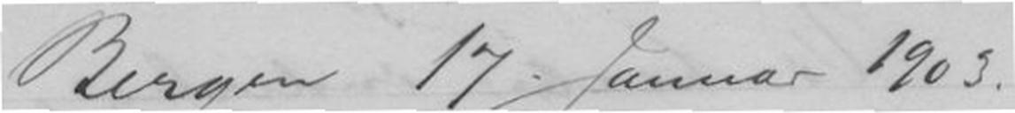Bergen 17 Januar 1903.
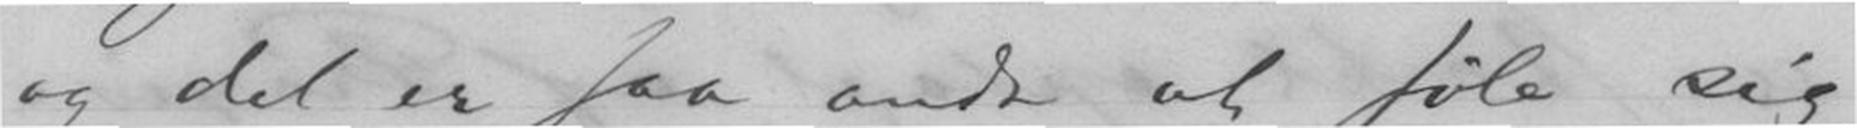og det er saa ondt at føle sig
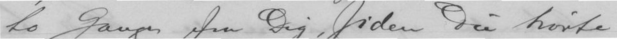to Gange fra Dig, siden Du hørte
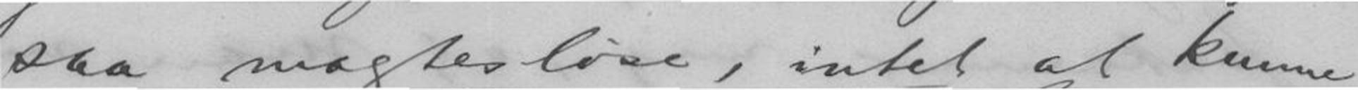saa magtesløse, intet at kunne
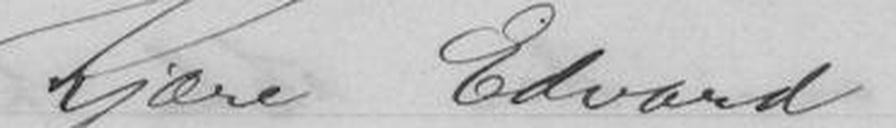Kjære Edvard!
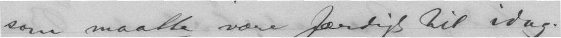som maatte være færdigt til idag.
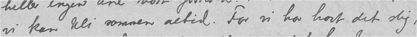vi kan bli sammen altid. For vi har havt det slig,
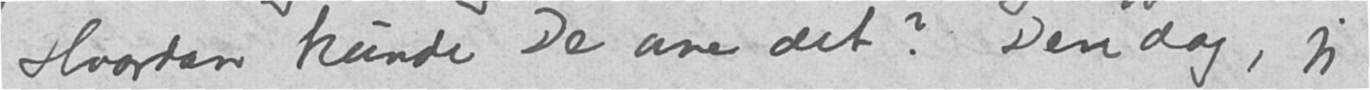Hvordan kunde De ane det? Den dag, jeg
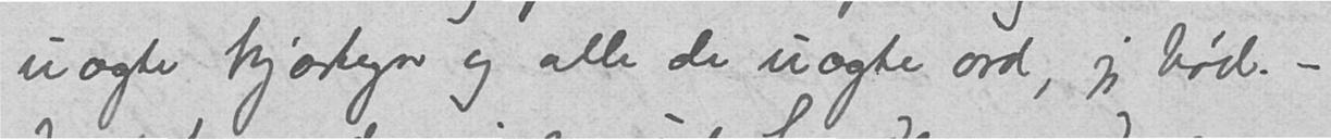uægte kjærtegn og alle de uægte ord, jeg bød. -
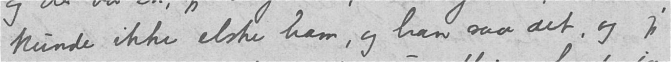kunde ikke elske ham, og han saa det, og jeg
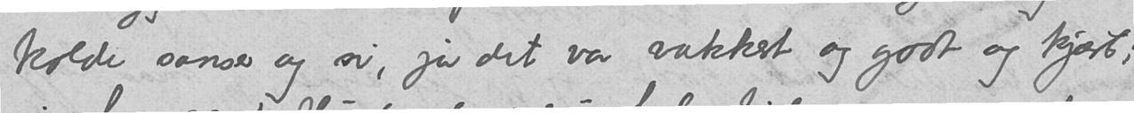kolde sanser og si, ja det var vakkert og godt og kjært;
